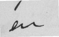en
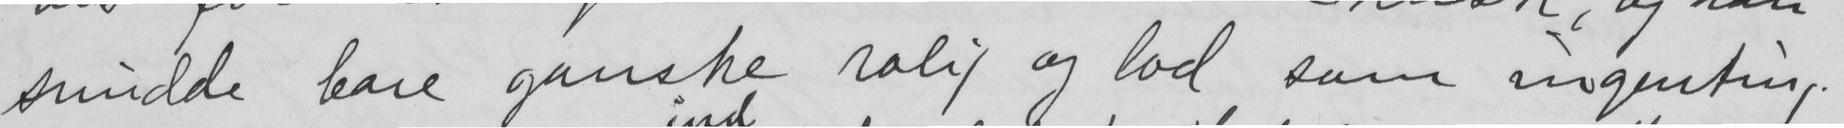snudde bare ganske rolig og lod som ingenting.
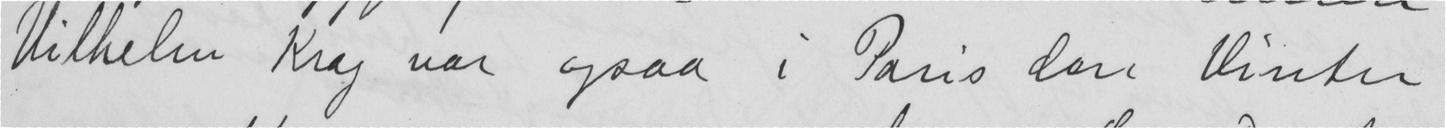Vilhelm Krag var ogsaa i Paris den Vinter
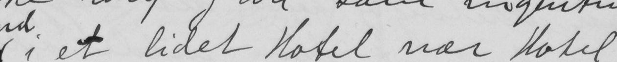i et lidet Hotel nær Hotel
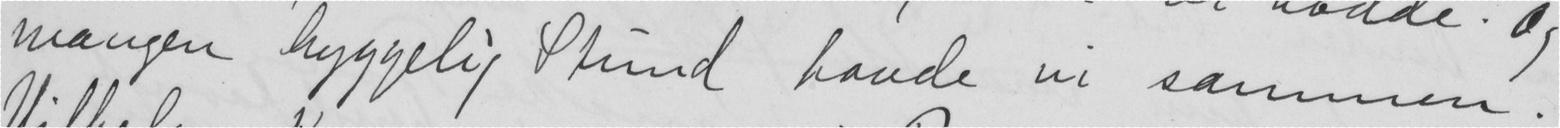mangen hyggelig Stund havde vi sammen.
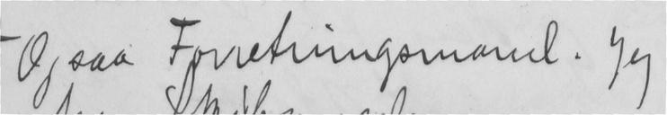Ogsaa Forretningsmand. Jeg
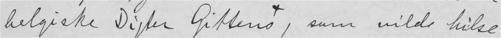helgiske Digter Gittens, som vilde hilse
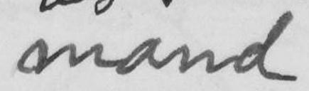mand
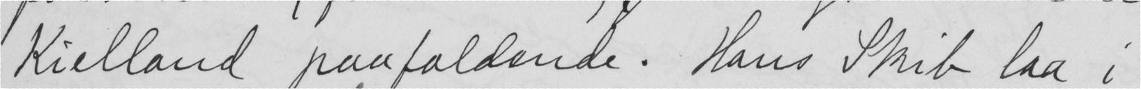Kielland paafaldende. Hans Skib laa i
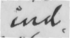ind
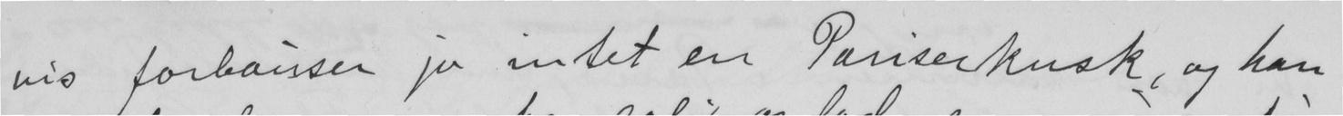vis forbauser jo intet en Pariserkusk, og han
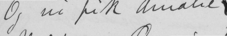Og vi fik Amalie
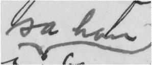sa han
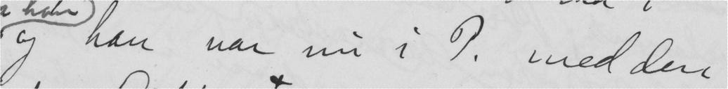og han var nu i P. med den
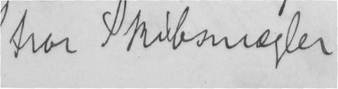tror Skibsmægler
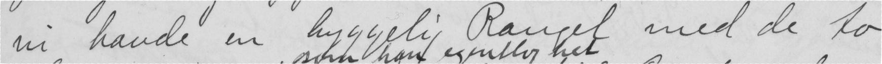vi havde en hyggelig Rangel med de to
